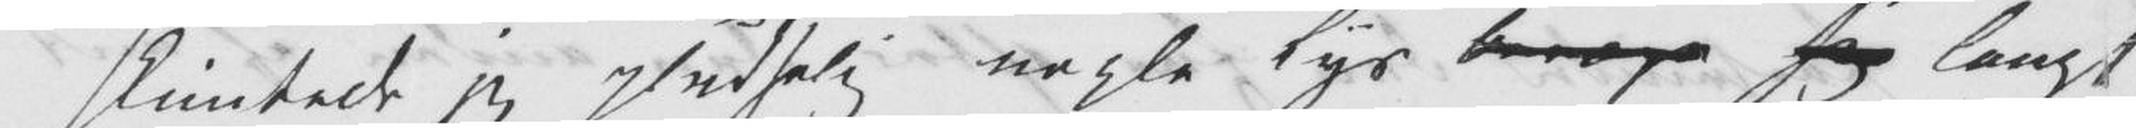skimtede jeg pludselig nogle Lys bevæge sig langt
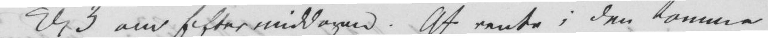Kl 3 om Eftermiddagen. At vente i den tomme
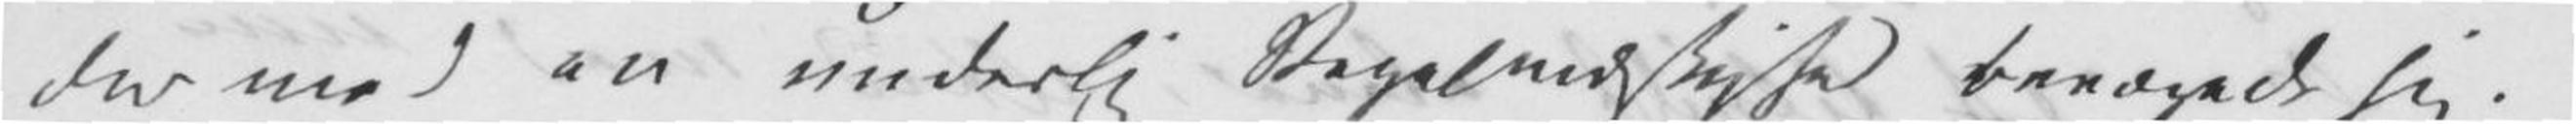der med en underlig Regelmæssighed bevægede sig.
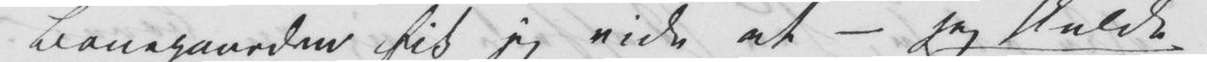Banegaarden fik jeg vide at – jeg skulde
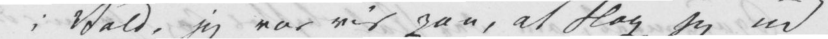i Vold, jeg var vis paa, at slap jeg ud
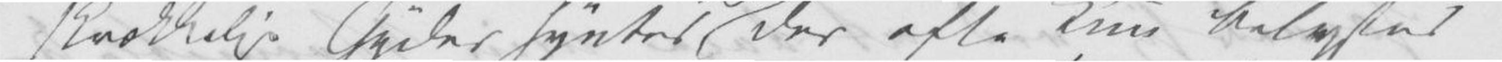skrækkelige Gyder syntes jeg der ofte kun belystes
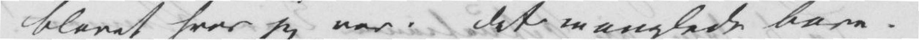blevet hvor jeg var. Det manglede bare.
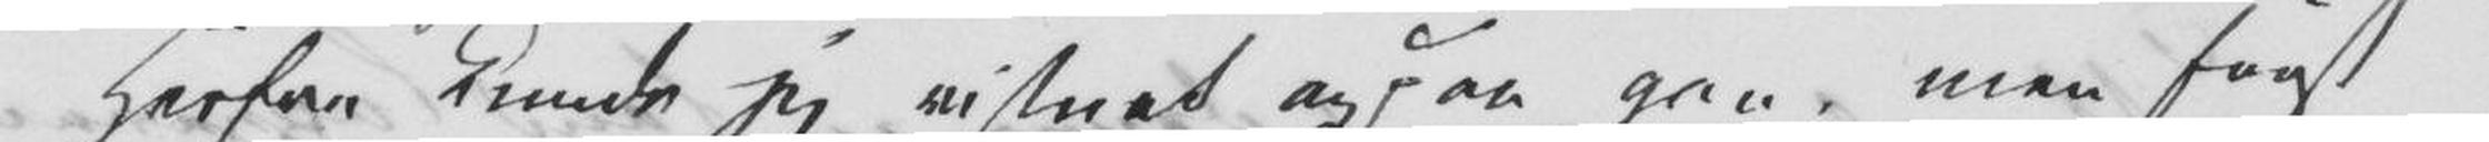Herfra kunde jeg vistnok ogsaa gaa, men først
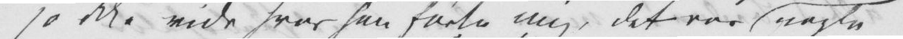jo ikke vide hvor han førte mig, det var nogle
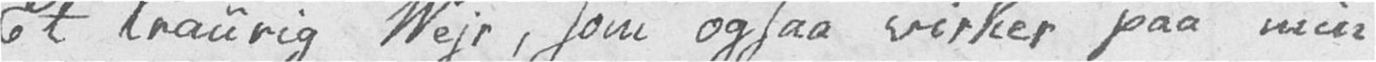Et traurig Vejr, som ogsaa virker paa min
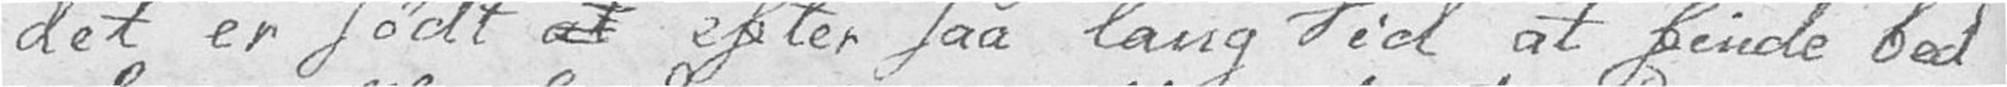det er sødt at efter saa lang Tid at finde bed
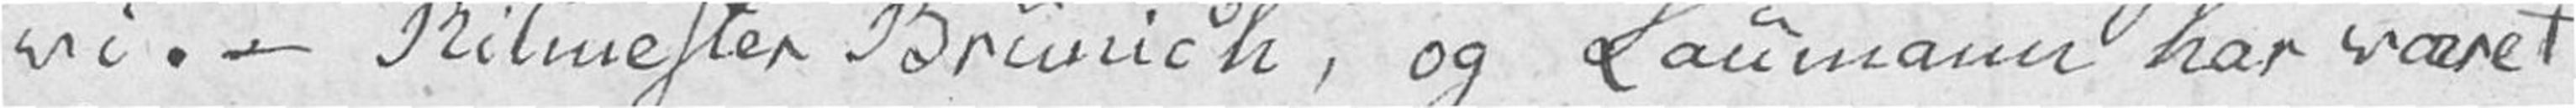vi. – Ritmester Brunich, og Laumann har været
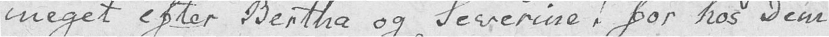meget efter Bertha og Severine! for hos Dem
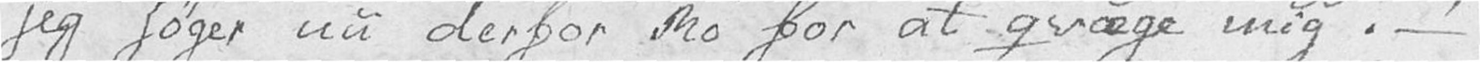jeg søger nu derfor Ro for at qvæge mig. –
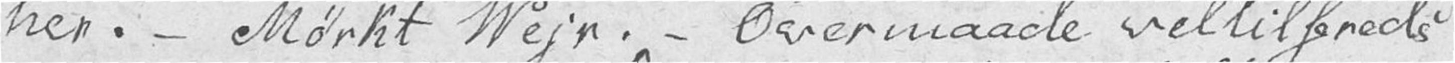her. – Mørkt Vejr. – Overmaade veltilfreds
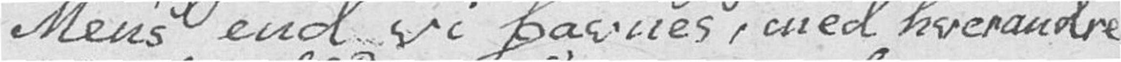Mens end vi favnes, med hverandre
